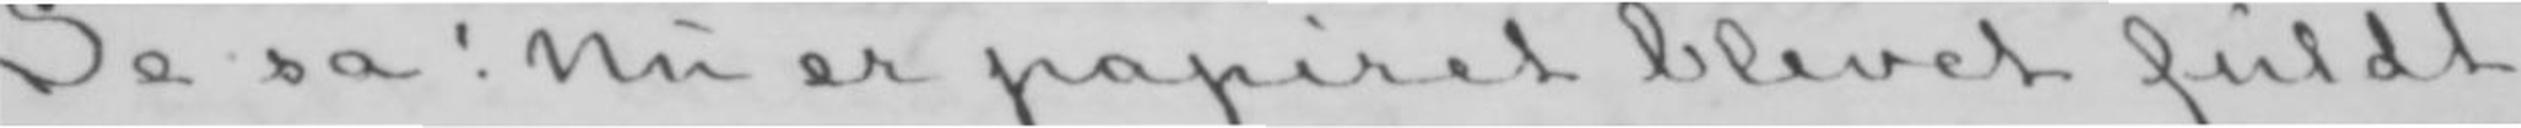Se så! Nu er papiret blevet fuldt
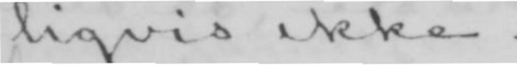ligvis ikke.
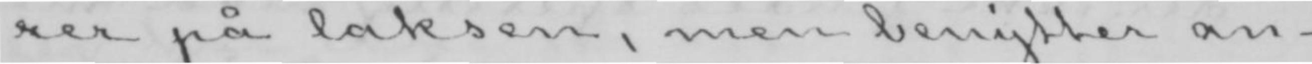rer på laksen, men benytter an-
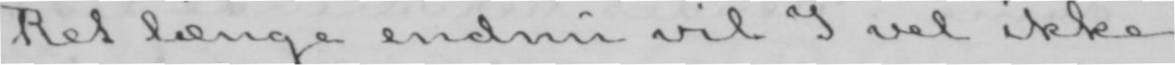Ret længe endnu vil I vel ikke
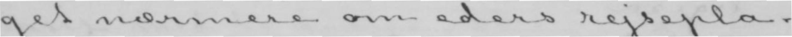get nærmere om eders rejsepla-
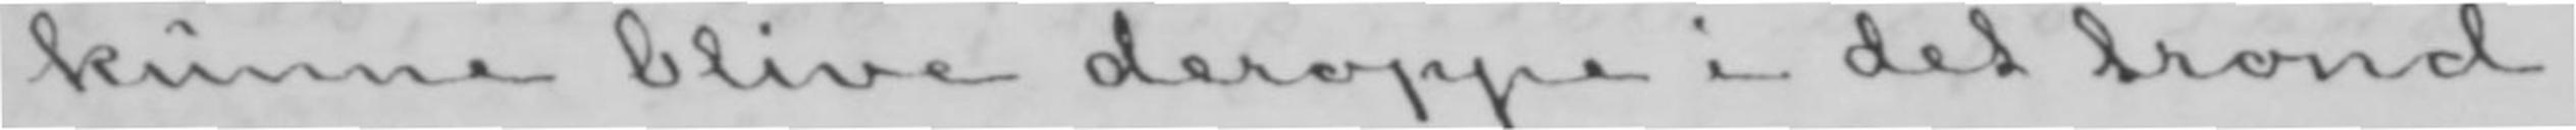kunne blive deroppe i det trond-
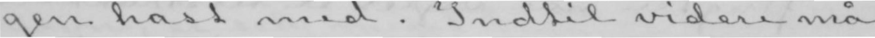gen hast med. Indtil videre må
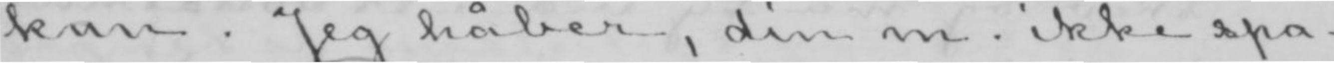kan. Jeg håber, din m. ikke spa-
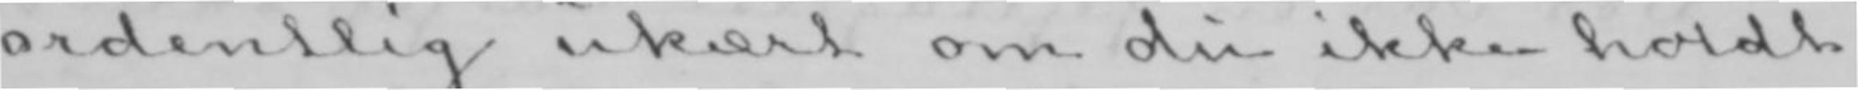ordentlig ukært om du ikke holdt
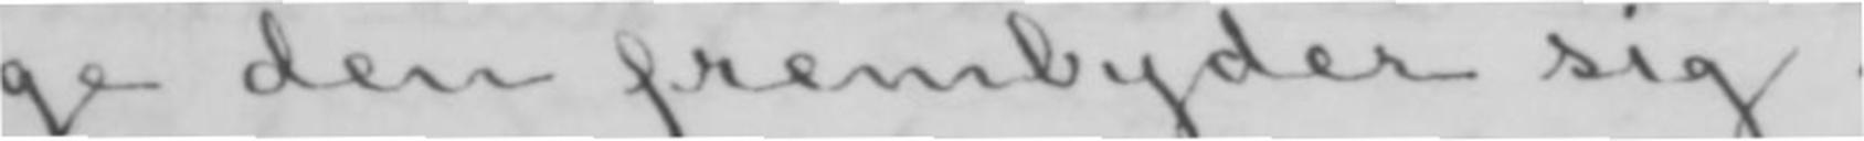ge den frembyder sig.
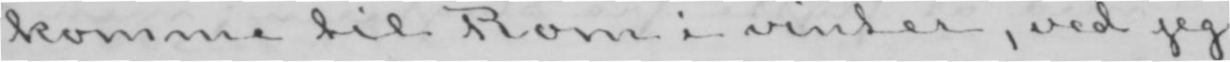komme til Rom i vinter, ved jeg
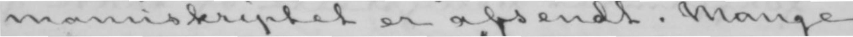manuskriptet er afsendt. Mange
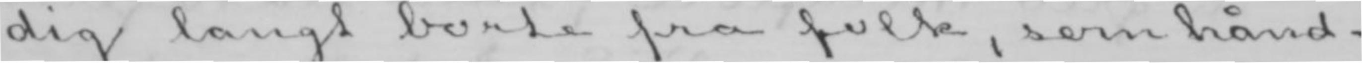dig langt borte fra folk, som hånd-
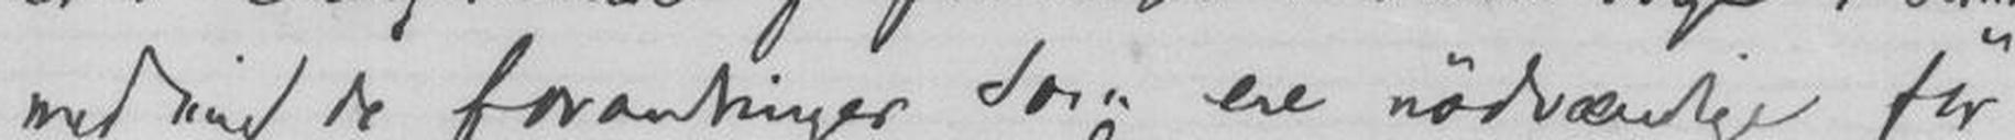med mig de forandringer som ere nödvændige för
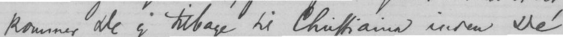kommer De ej tilbage til Christiania inden Dé
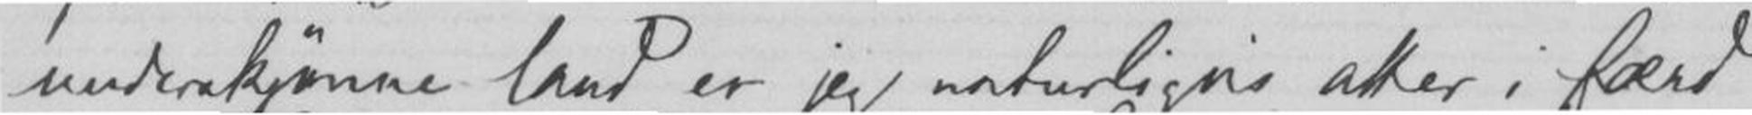underskjönne land, er jeg naturligvis atter i færd
